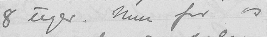8 uger. Men for os
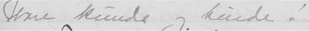bare kunde og burde!
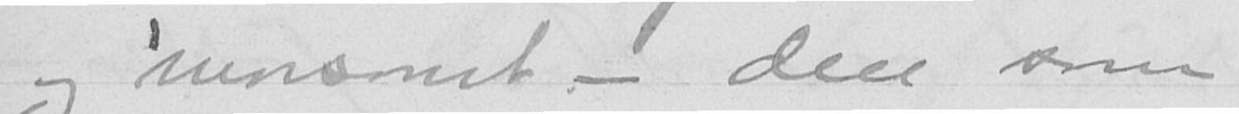og morsomt - den som
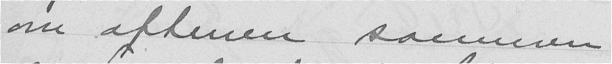om aftenen sammen
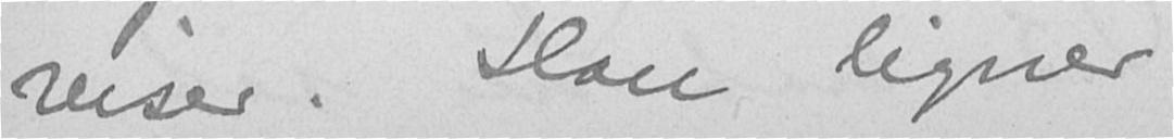reiser. Han ligner
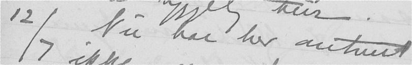12/7 Nu har her omtrent
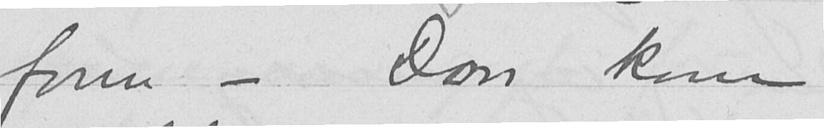form. - Don kom
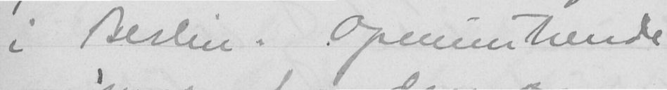i Berlin. Opmundtrende
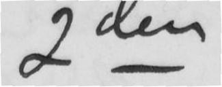2den
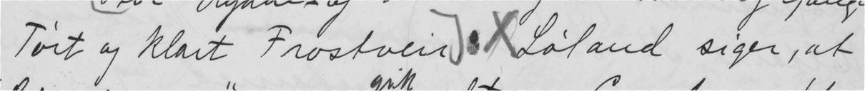Tørt og klart Frostveir. Løland siger, at
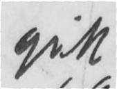gik
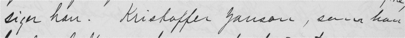siger han. Kristoffer Janson, som han
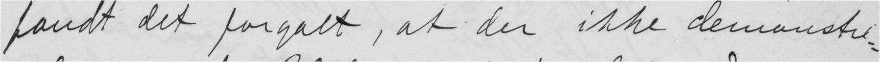fandt det forgalt, at der ikke demonstre-
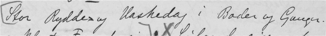Stor Rydde og Vaskedag i Boder og Ganger.
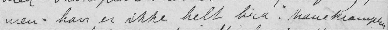men han er ikke helt bra. Mavekramperne
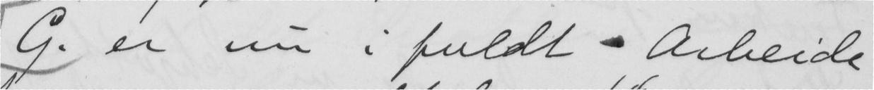G. er nu i fuldt Arbeide
